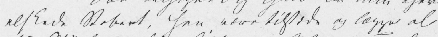elskede Robert, han være tilstæde og lægge al
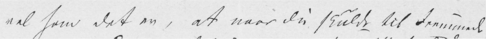vel som det er, at naar Du skulde til Fremmede
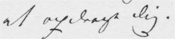at opdrage Dig.
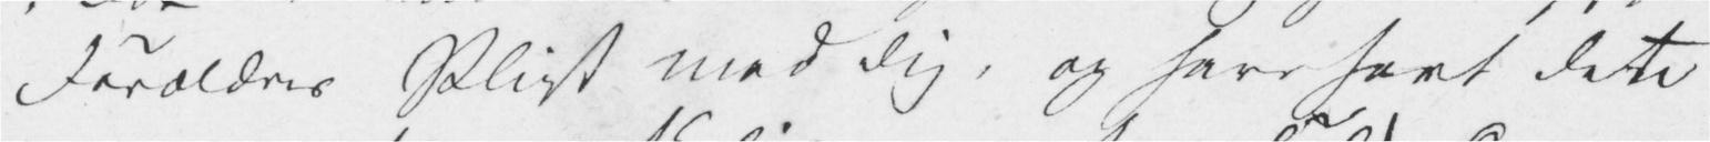Forældres Pligt mod Dig, og have havt den
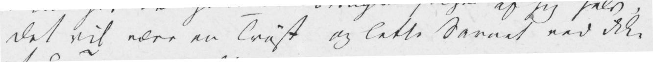Det vil være en Trøst og lette Savnet ved ikke
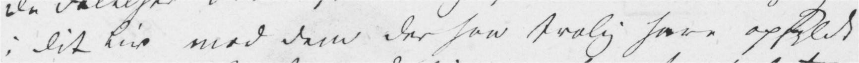i Dit Liv mod dem der saa trolig have opfyldt
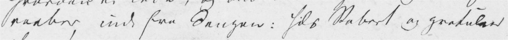raaber inde fra Sengen: Hils Robert og gratuleer
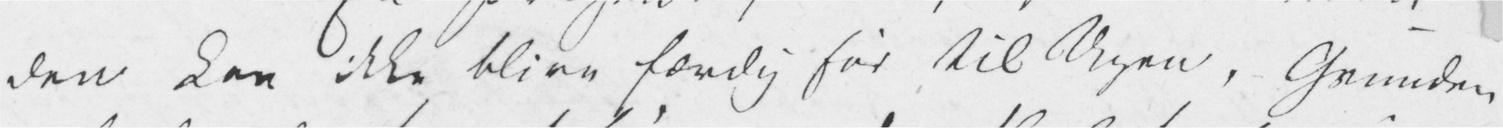den kan ikke blive færdig før til Ugen, Grunden
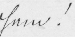ham!
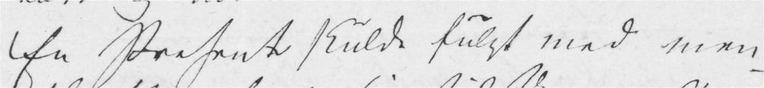En Present skulde fulgt med men
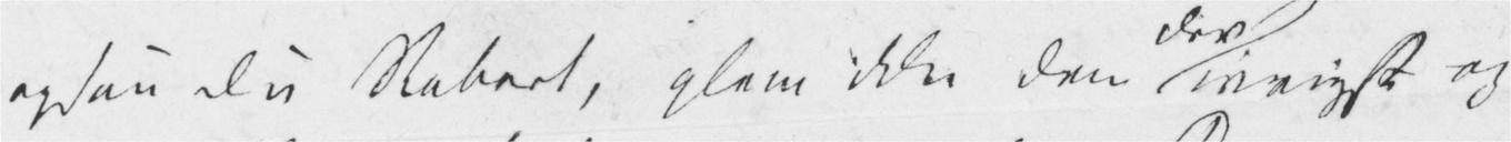ogsaa Du Robert, glem ikke den ivrigst og
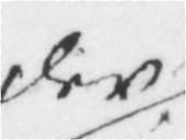der
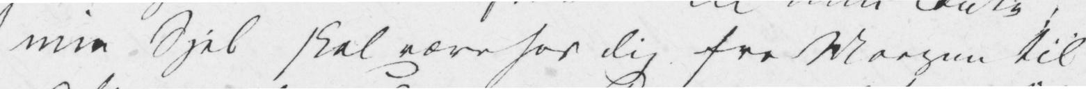min Sjel skal være hos Dig fra Morgen til
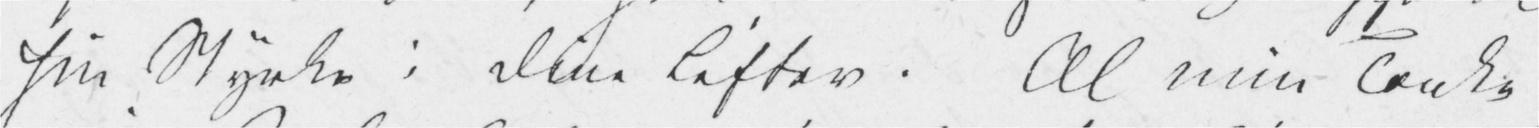sin Styrke i Dine Løfter. Al min Tanke,
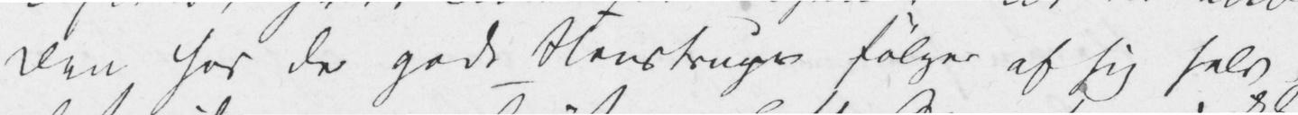den hos de gode Stenstrups følger af sig selv.
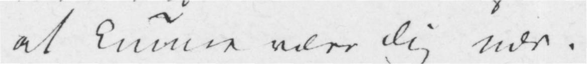at kunne være Dig nær.
